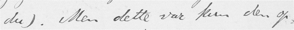du). Men dette var kun den op-
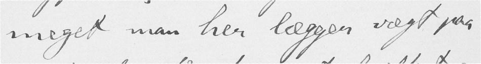meget man her lægger vægt paa
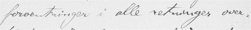forventninger i alle retninger over-
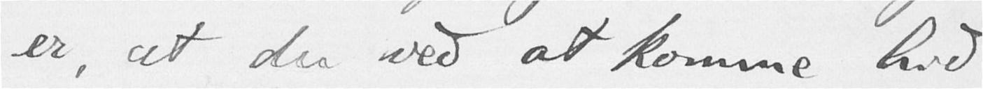er, at du ved at komme hid
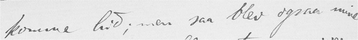komme hid; men saa blev ogsaa mine
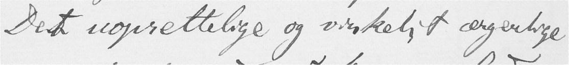Det uoprettelige og virkeligt ærgerlige
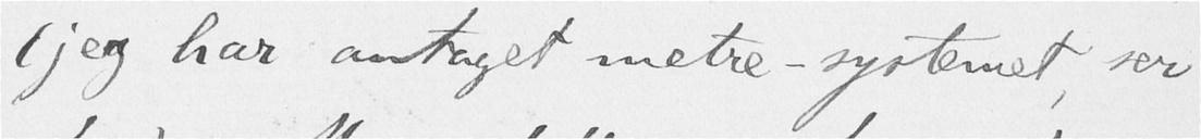(jeg har antaget metre-systemet, ser
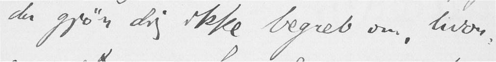du gjør dig ikke begreb om, hvor-
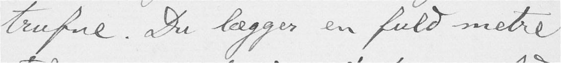trufne. Du lægger en fuld metre
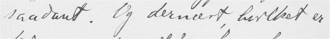saadant. Og dernæst, hvilket er
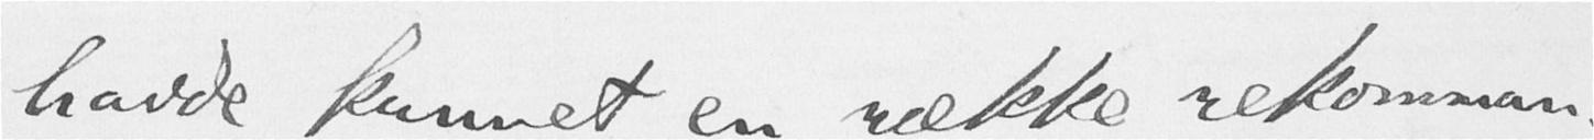havde kunnet en række rekomman-
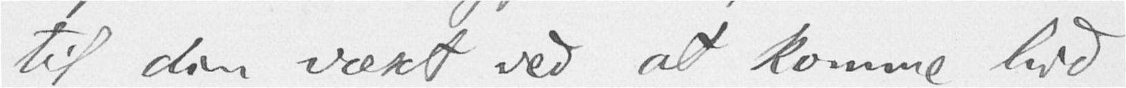til din væxt ved at komme hid
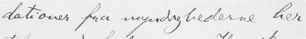dationer fra myndighederne her
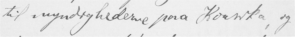til myndighederne paa Korsika, og
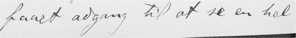faaet adgang til at se en hel
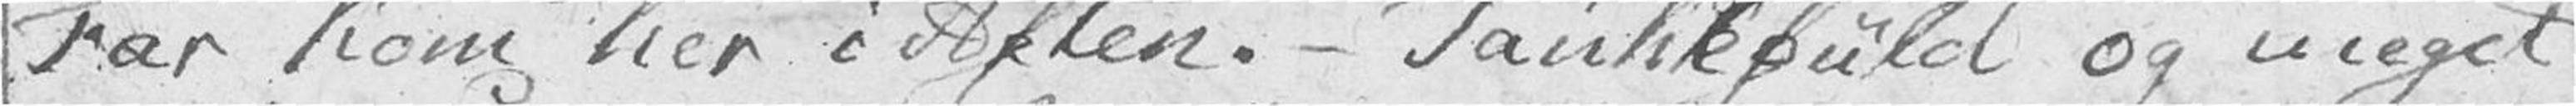Far kom her i Aften. – Tankefuld og meget
